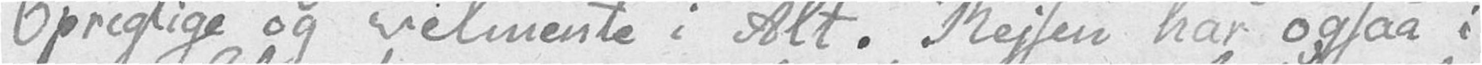Oprigtige og velmente i Alt. Rejsen har ogsaa i
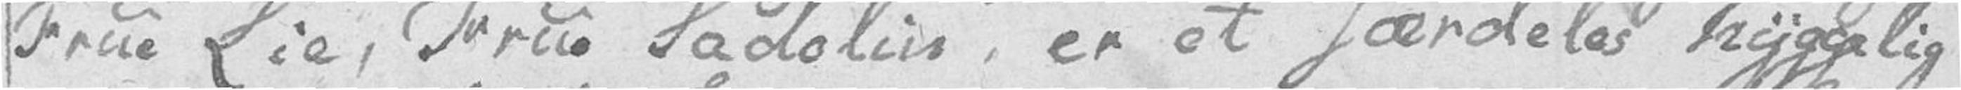Frue Lie, Frue Sadolin, er et særdeles hyggelig
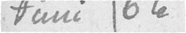Juni 6te
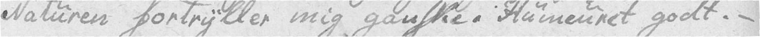Naturen fortryller mig ganske. Humeuret godt. –
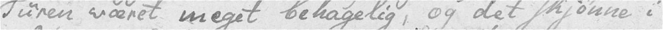Turen været meget behagelig, og det skjønne i
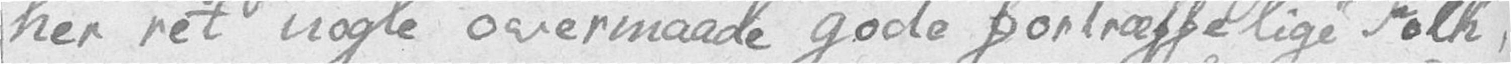her ret nogle overmaade gode fortræffelige Folk,
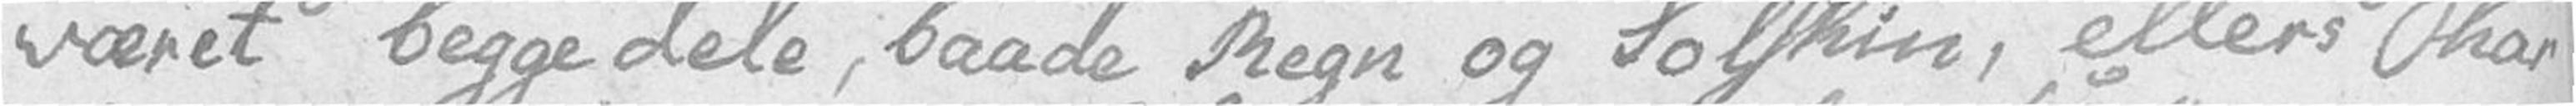været begge dele, baade Regn og Solskin, ellers har
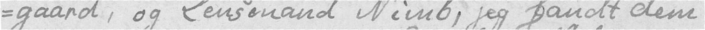-gaard, og Lensmand Nimb, jeg fandt dem
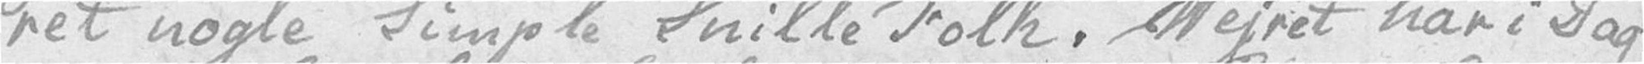ret nogle Simple Snille Folk. Vejret har i Dag
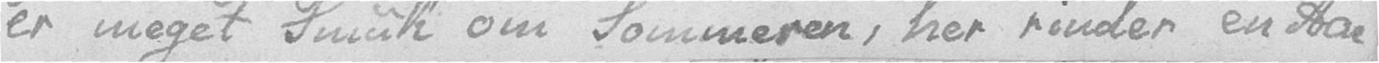er meget Smuk om Sommeren, her rinder en Aae
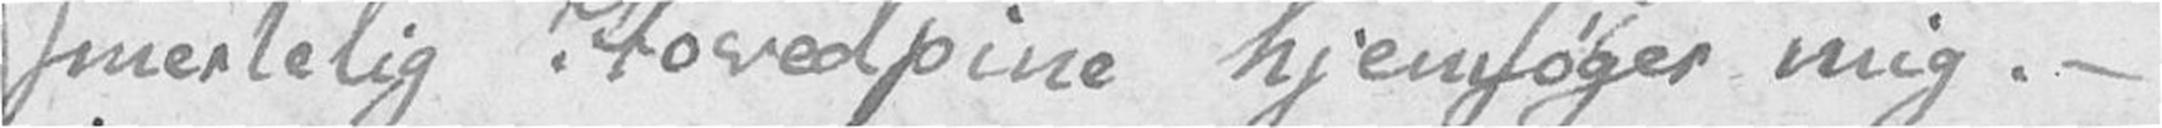smertelig Hovedpine hjemsøger mig. –
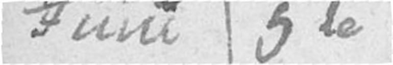Juni 5te
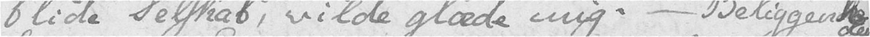blide Selskab, vilde glæde mig. – Beliggenheden
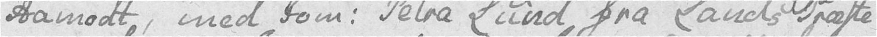Aamodt, med Jom: Petra Lund fra Lands Præste
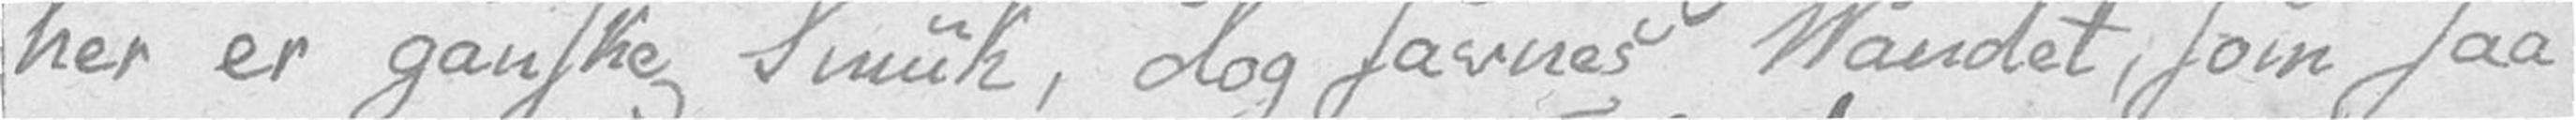her er ganske Smuk, dog savnes Vandet, som saa
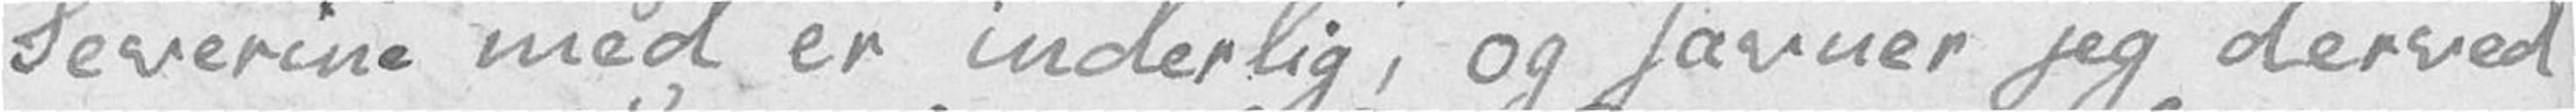Severine med er inderlig, og savner jeg derved
